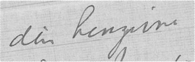din hengivne
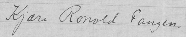Kjære Ronald Fangen,
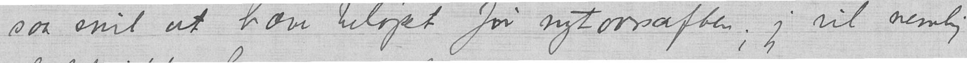saa snil at hæve beløpet før nytaarsaften; jeg vil nemlig
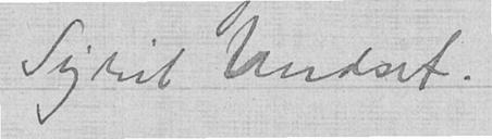Sigrid Undset.
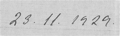23.11.1929.
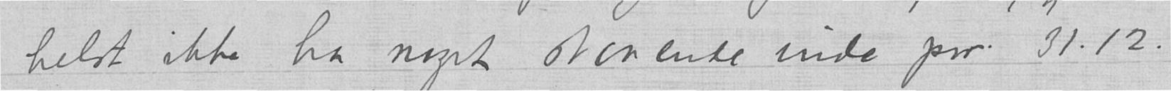helst ikke ha noget staaende inde per. 31.12.
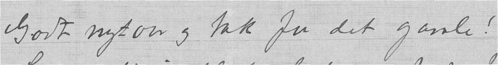Godt nytaar og tak for det gamle!
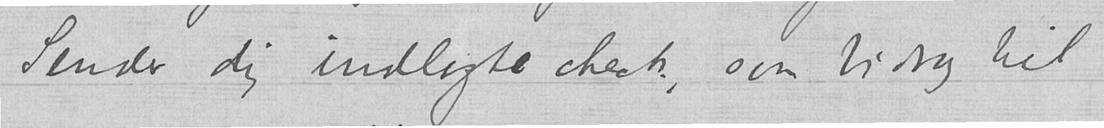Sender dig indlagte check, som bidrag til
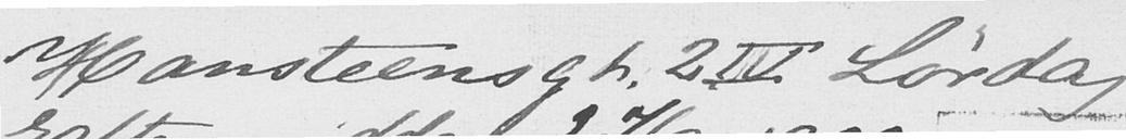Hansteensgt: 2 IV Lørdag
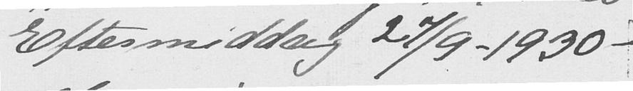Eftermiddalg 27/9-1930-
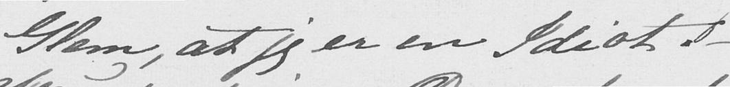Glem, at jeg er en Idiot!
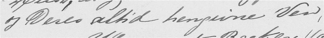og Deres altid hengivne Ven,
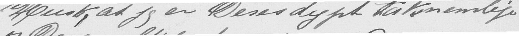Husk, at jeg er Deses dypt taknemlige
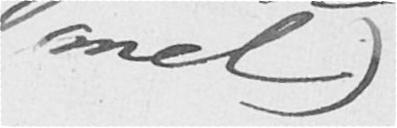mel
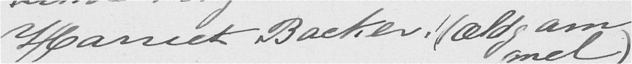Harriet Backer (ældgam-
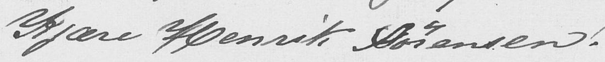Kjære Henrik Sørensen!
